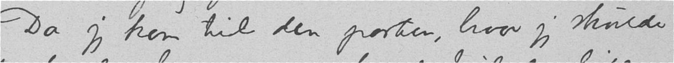Da jeg kom til den porten, hvor jeg skulde
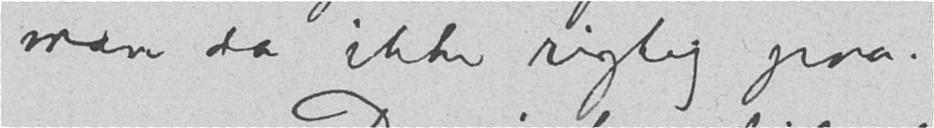man da ikke rigtig paa.
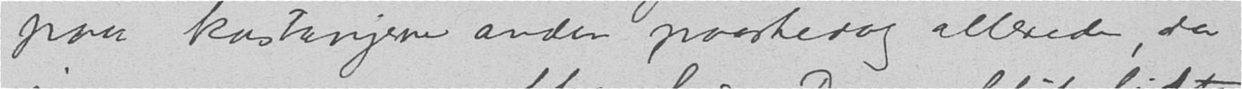paa kastanjerne anden paaskedag allerede da
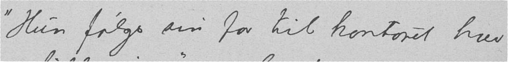"Hun følger sin far til kontoret hver
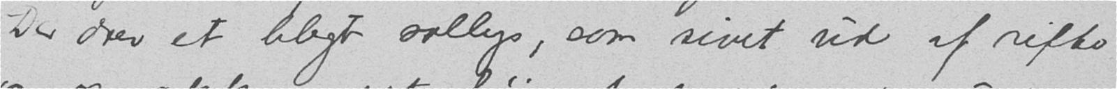Der drev et blegt sollys, som sivet ud af rifter
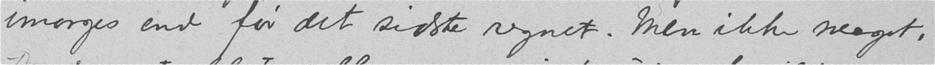imorges end før det sidste regnet. Men ikke meget.
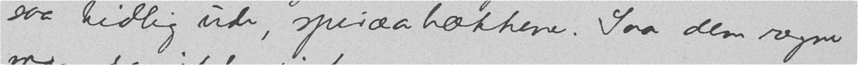saa tidlig ude, spiræahækkene. Saa dem regner
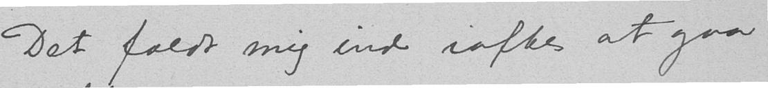Det faldt mig ind iaftes at gaa
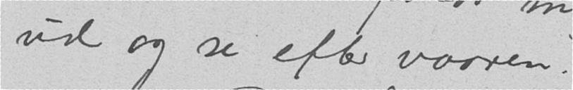ud og se efter vaaren.
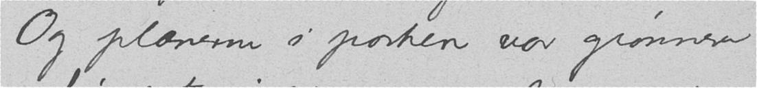Og plænerne i parken var grønnere
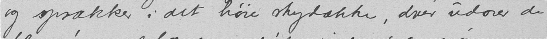og sprækker i det høie skydække, drev udover de
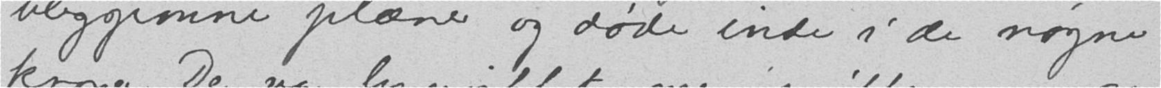bleggrønne plæner og døde inde i de nøgne
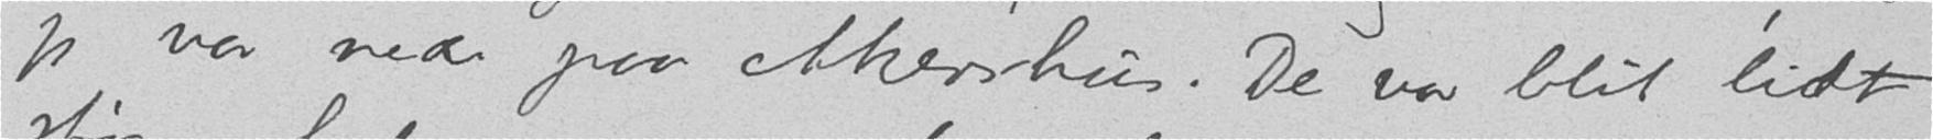jeg var nede paa Akershus. De var blit lidt
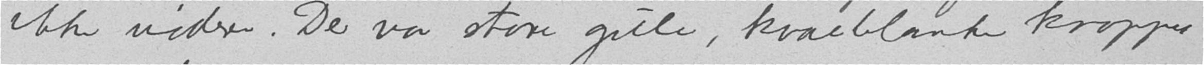ikke videre. Der var store gule, kvaeblanke knopper
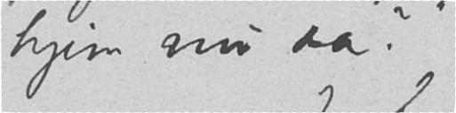hjem nu da?!"
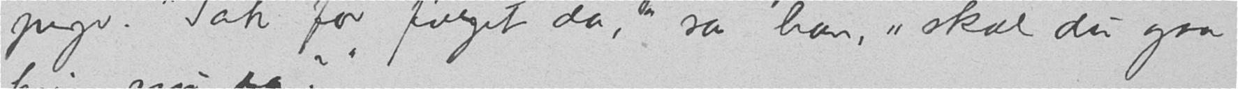pige. "Tak for følget da," sa han, "skal du gaa
